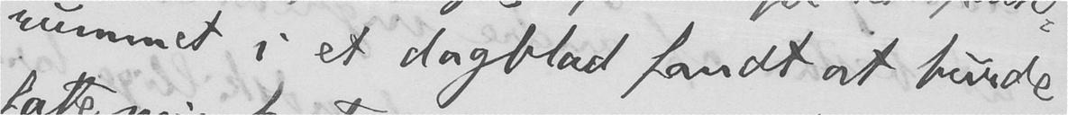rummet i et dagblad fandt at burde
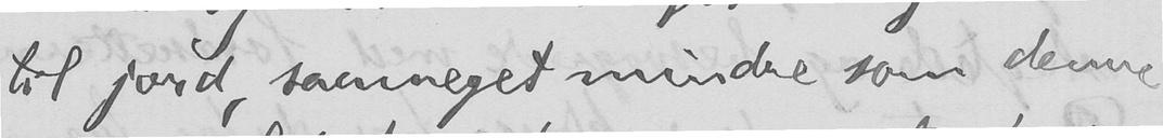til jord, saameget mindre som denne
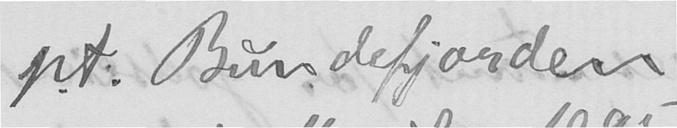pt. Bundefjorden
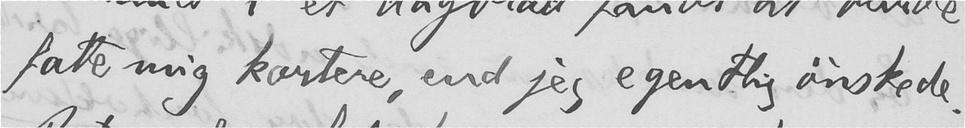fatte mig kortere, end jeg egentlig ønskede.
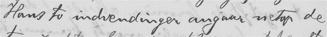Hans to indvendinger angaar netop de
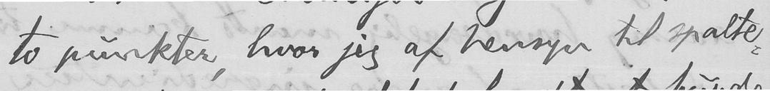to punkter, hvor jeg af hensyn til spalte-
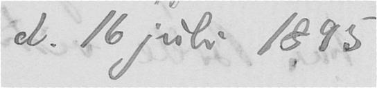d. 16 juli 1895.
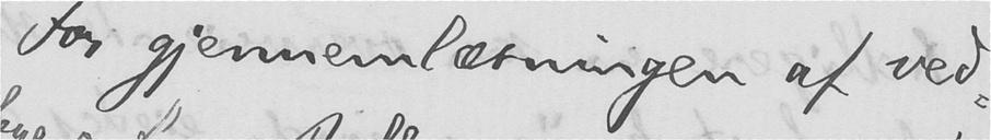For gjennemlæsningen af ved-
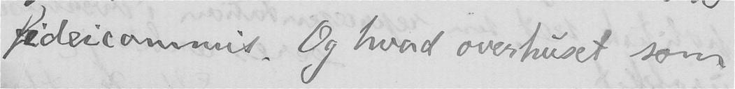fideicommis. Og hvad overhuset som
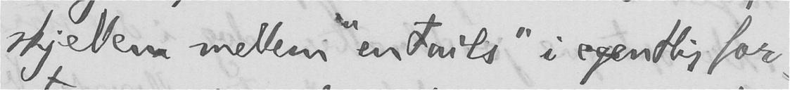skjellen mellem "entails" i egentlig for-
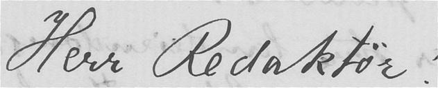Herr Redaktør!
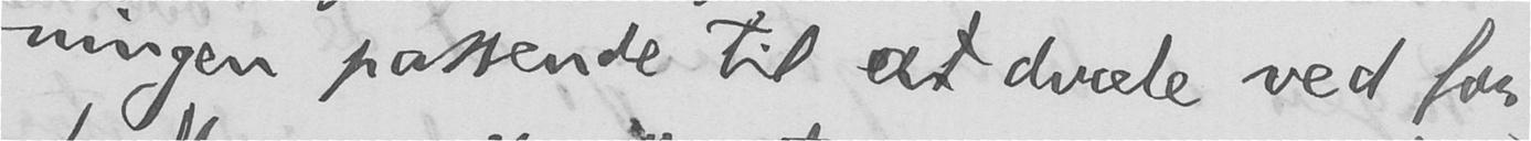ningen passende til at dvæle ved for-
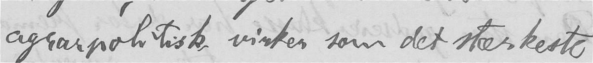agrarpolitisk virker som det stærkeste
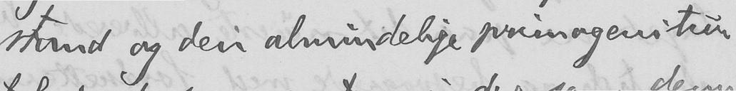stand og den almindelige primogenitur
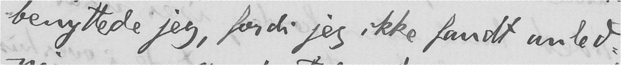benyttede jeg, fordi jeg ikke fandt anled-
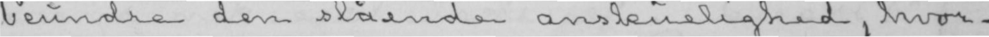beundre den slående anskuelighed, hvor-
